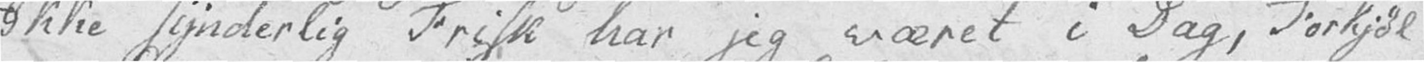Ikke synderlig Frisk har jeg været i Dag, Forkjøl
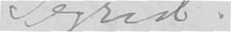Sigrid
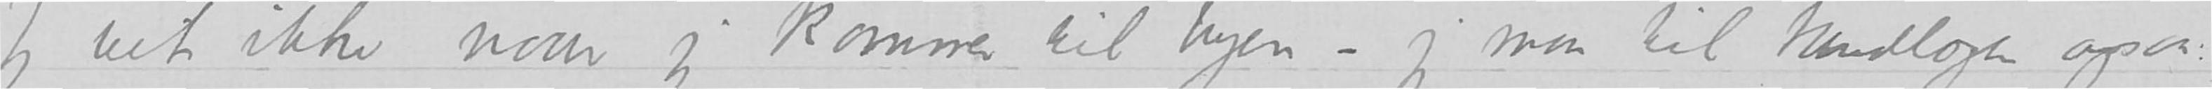Jeg vet ikke naar jeg kommer til byen- jeg maa til tandlægen ogsaa
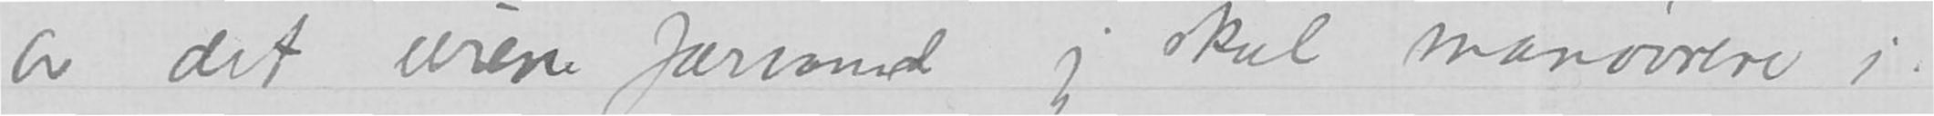av det urene farvand jeg skal manøvrere i.
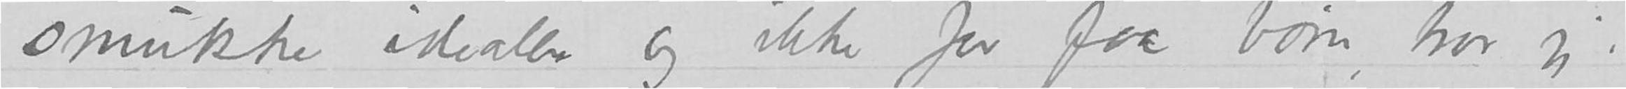smukke idealer og ikke for faa børn, tror jeg.
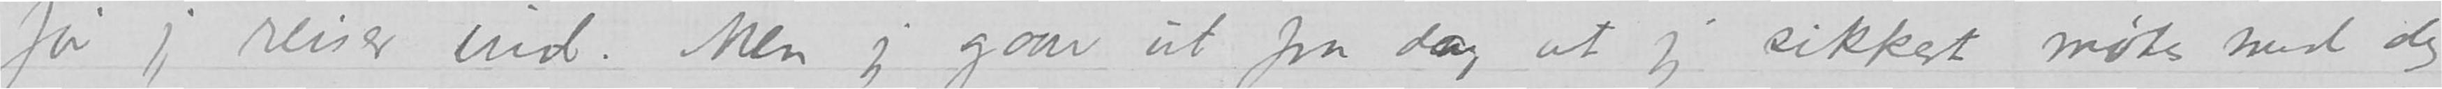før jeg reiser ind. Men jeg gaar ut fra da , at jeg sikkert møtes med dig –
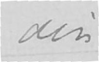din
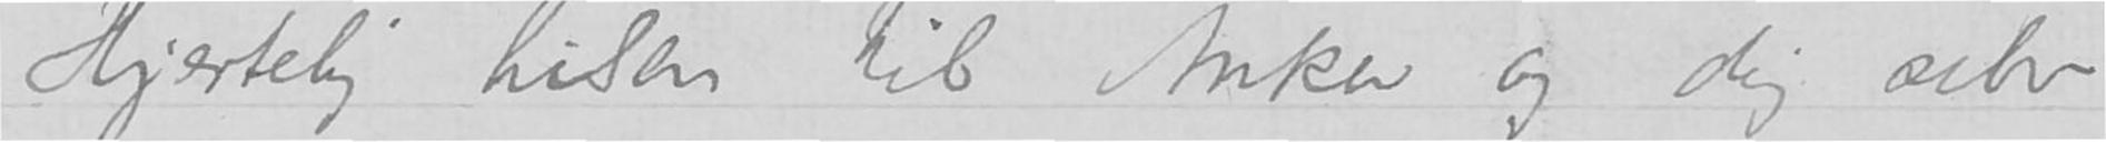Hjertelig hilsen til Anker og dig selv,
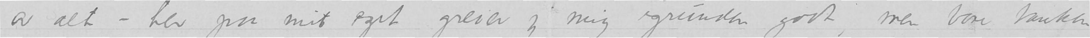av alt – her paa mit eget greier jeg mig igrunden godt, men bare tanken
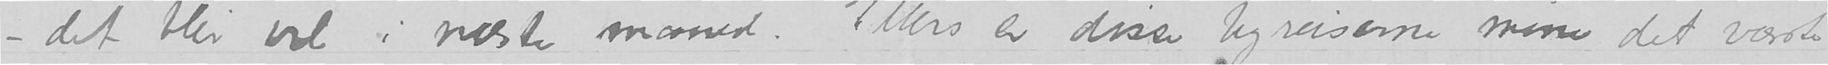det blir vel i neste maaned.  Ellers er disse byreiserne mine det værste
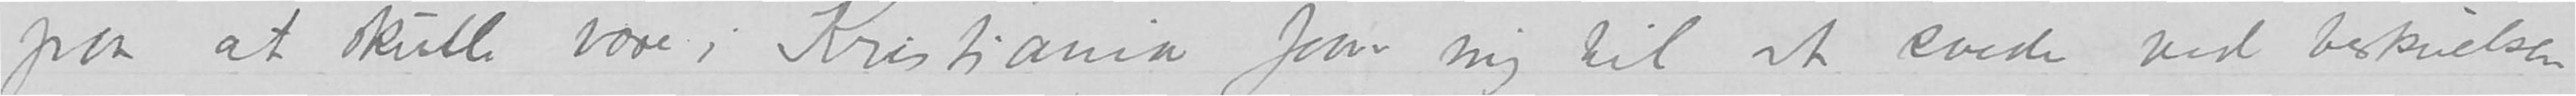paa at skulde være i Kristiania faar mig til at svede ved beskuelsen
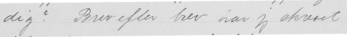dig? Brev efter brev har jeg skrevet
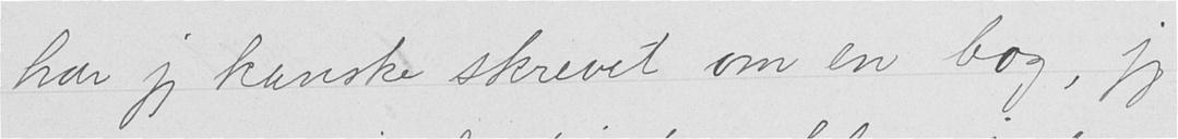har jeg kanske skrevet om en bog, jeg
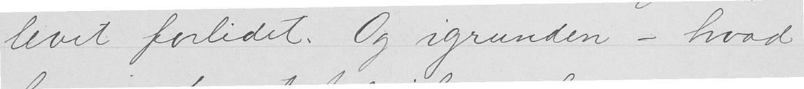levet forlidet. Og igrunden - hvad
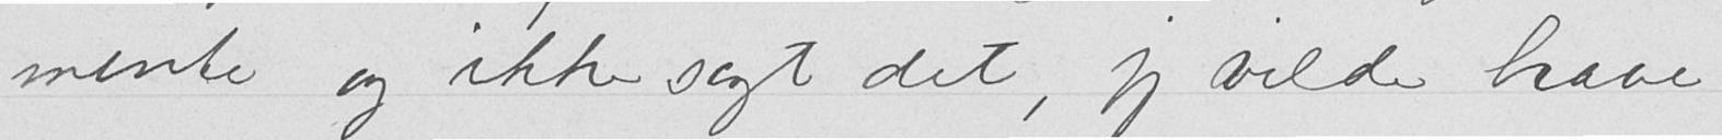mente og ikke sagt det, jeg vilde have
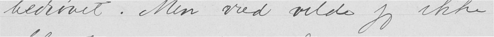bedrøvet. Men vred vilde jeg ikke
